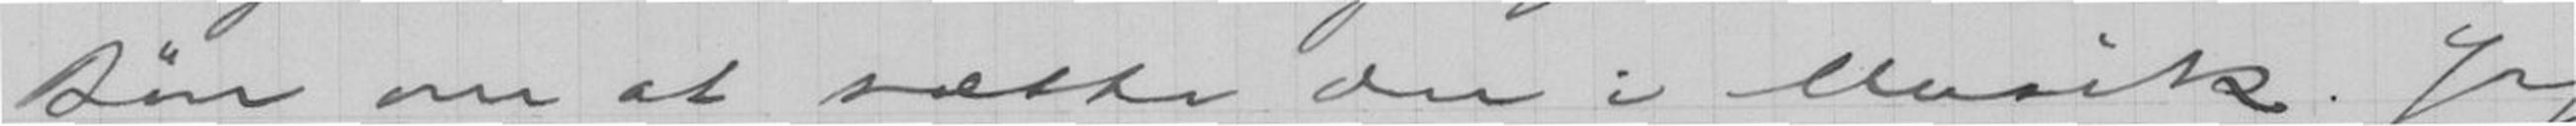Bøn om at sætte den i Musik. Jeg
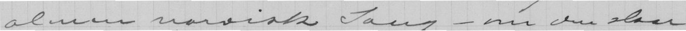almen nordisk Sang - om den slaar
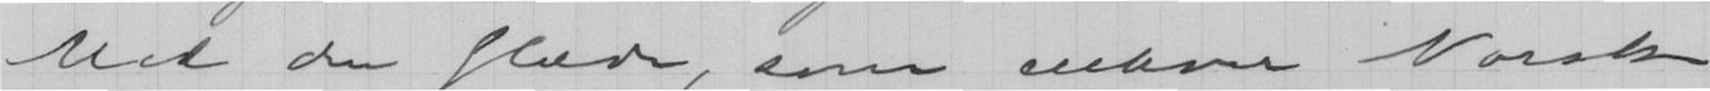Med den glede, som enhver Norsk
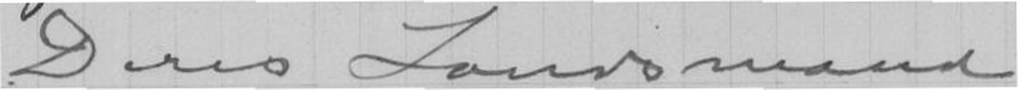Deres Landsmand
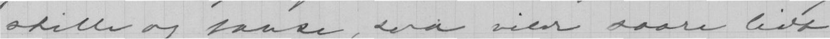stille og tause, saa vilde saare lidt
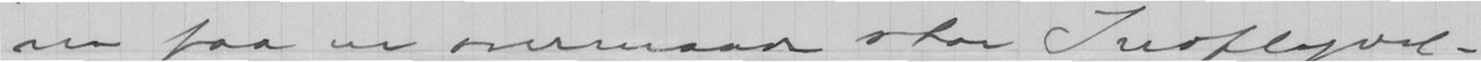ne faa en overmaade stor Indflydel-
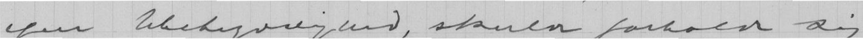egen Ubetydelighed, skulde forholde sig
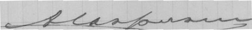A Caspersen
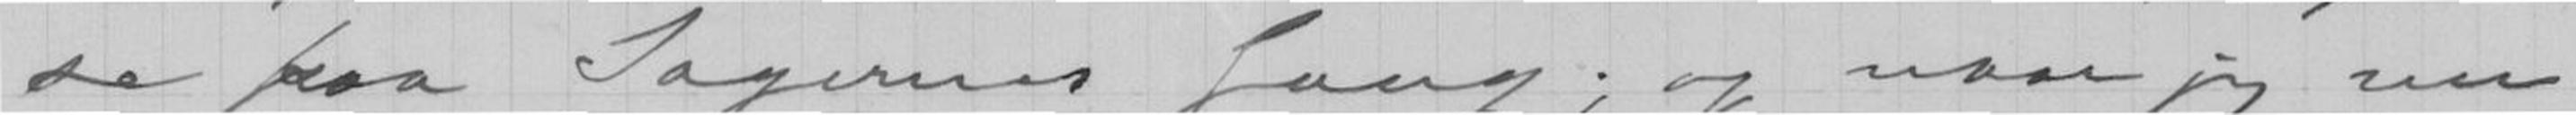se paa Sagernes Gang; og naar jeg nu
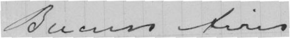Buenos Aires
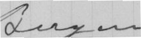Bergen
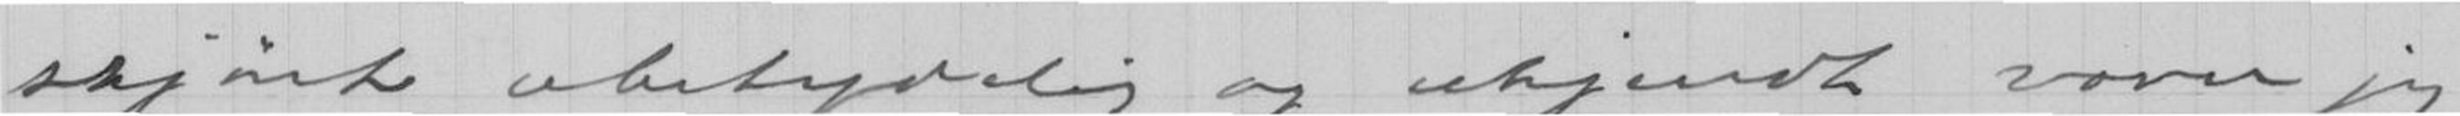skjønt ubetydelig og ukjendt vover jeg
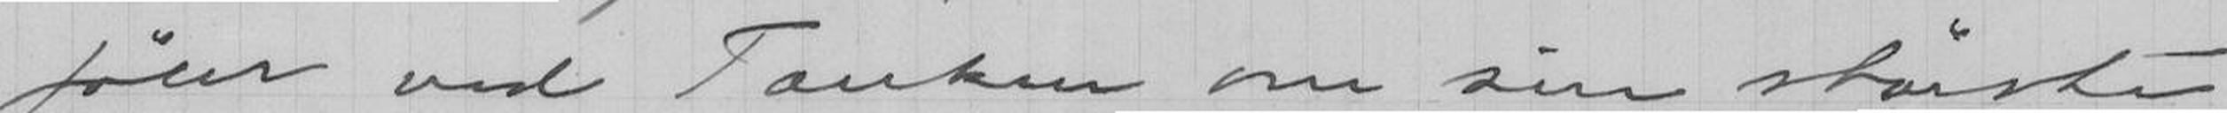føler ved Tanken om sin største
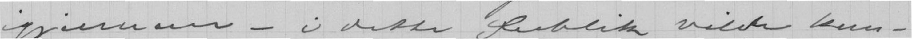igjennem - i dette Øieblik vilde kun-
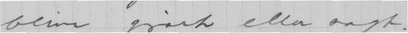blive gjort eller sagt.
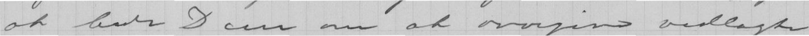at bede Dem om at overgive vedlagte
